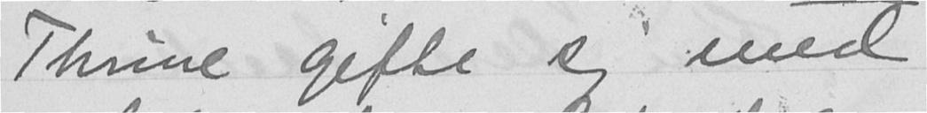Thrine gifte sig med
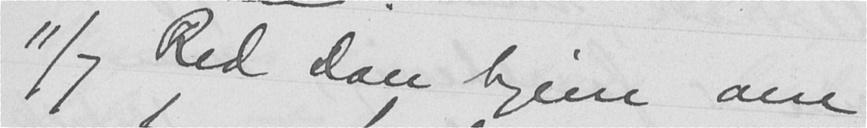11/7 Red Don hjem om
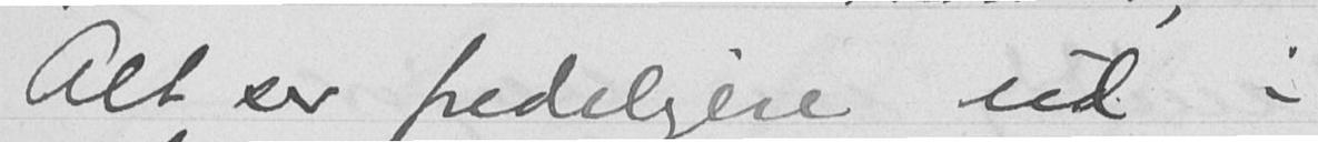Alt ser fredeligere ud i
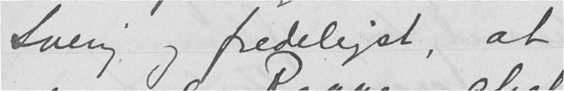Sverig og fredeligst, at
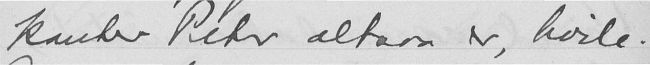kanter Peter altsaa er, hvile.
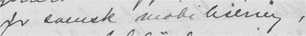for svensk mobilisering,
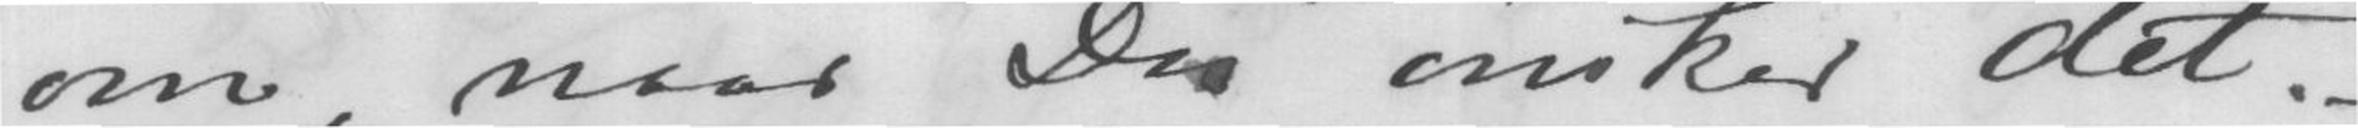om, naar Du ønsker det.-
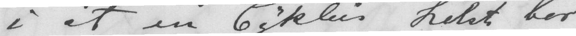i at en Dyklus helst bør
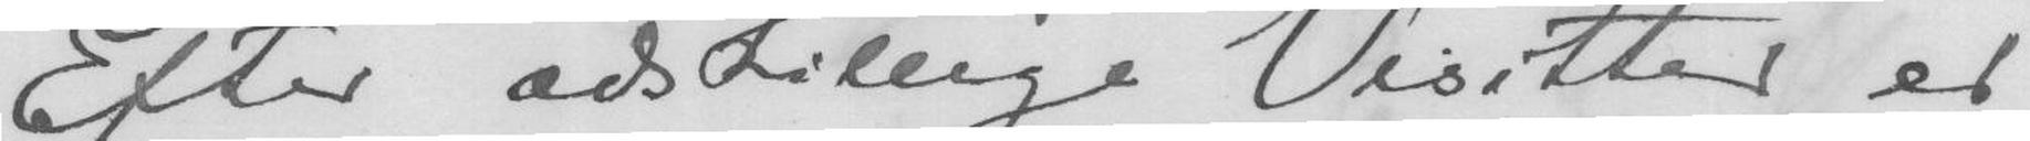Efter adskillige Visitter er
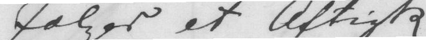følger et Aftryk
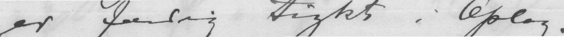er ferdig trygt Oplag.
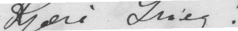Kjære Grieg!
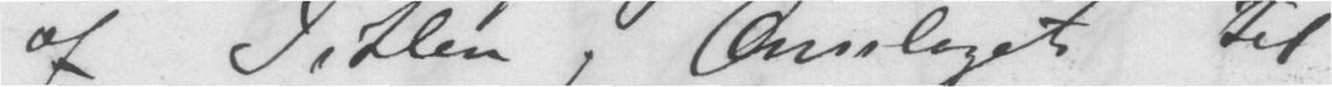af Titlen og Omlaget til
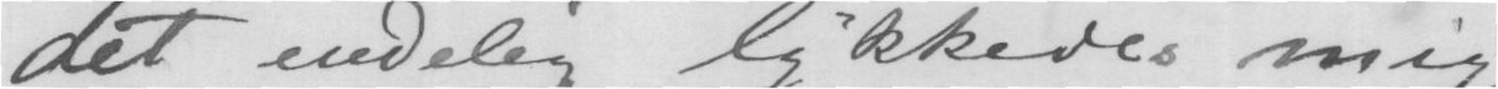det endelig lykkedes mig
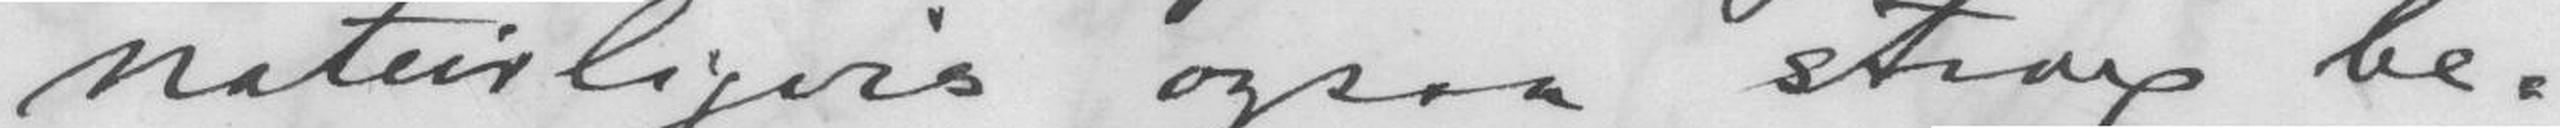naturligvis ogsaa strag be-
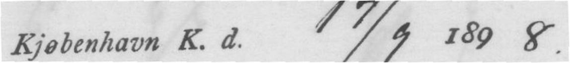Kjøbenhavn K.d. 17/9 1898
